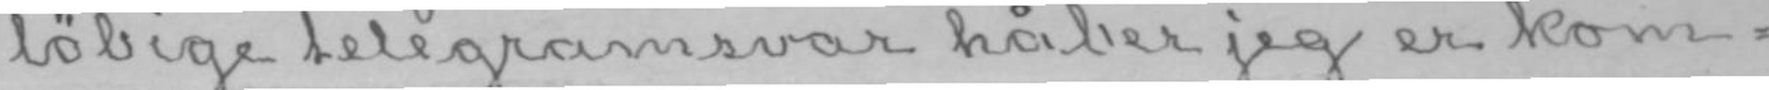løbige telegramsvar håber jeg er kom-
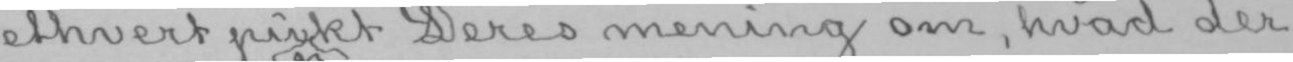ethvert punkt Deres mening om, hvad der
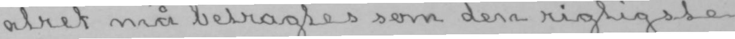atret må betragtes som den rigtigste
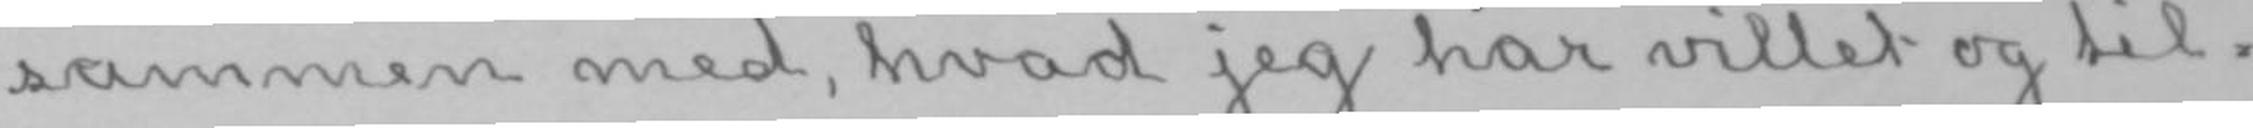sammen med, hvad jeg har villet og til-
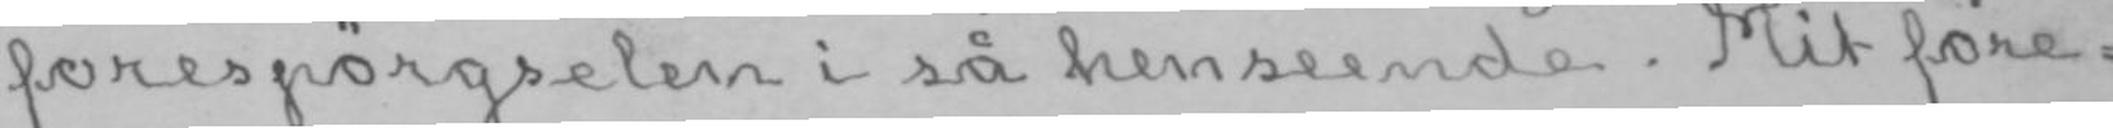forespørgselen i så henseende. Mit fore-
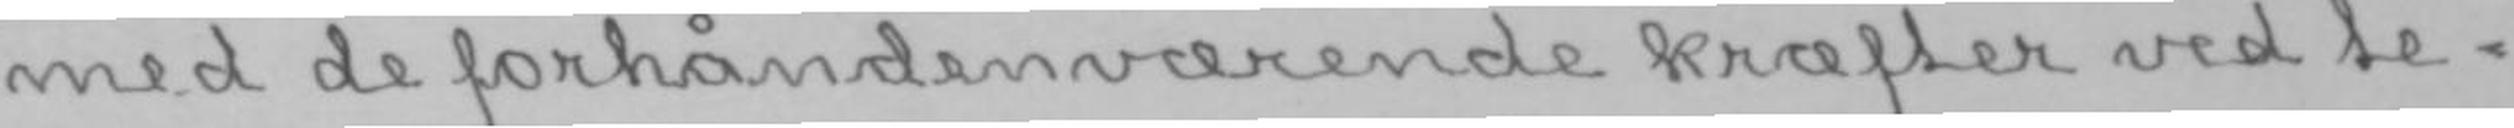med de forhåndenværende kræfter ved te-
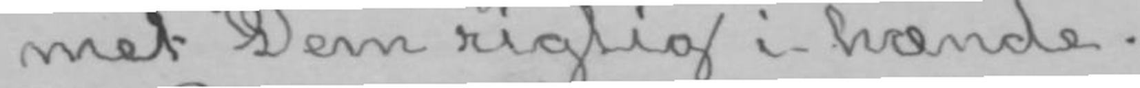met Dem rigtig i hænde.
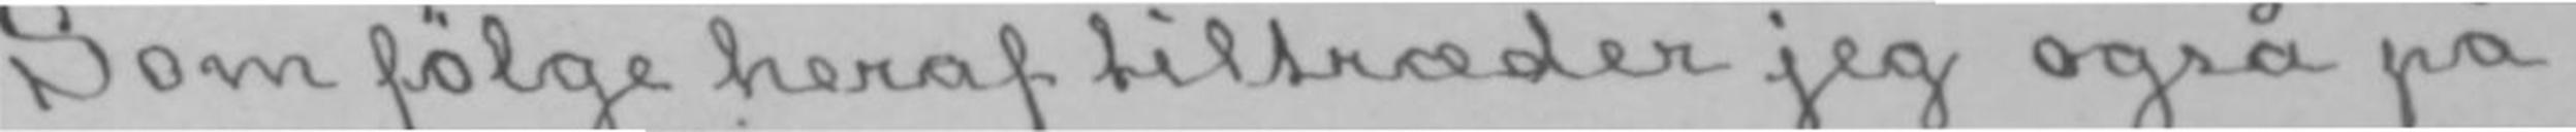Som følge heraf tiltræder jeg også på
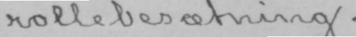rollebesætning.
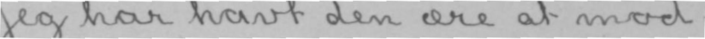Jeg har havt den ære at mod-
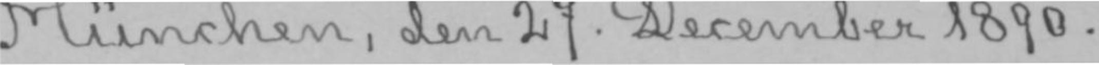München, den 27. December 1890.
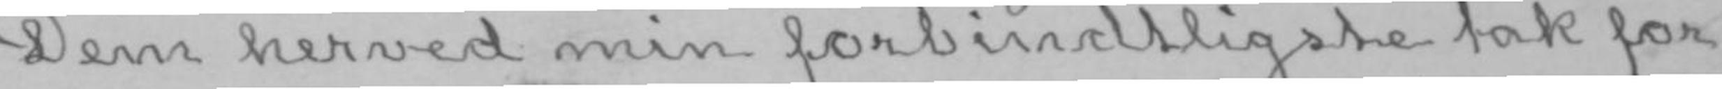Dem herved min forbindtligste tak for
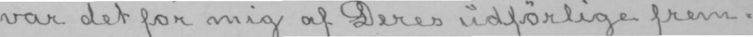var det for mig af Deres udførlige frem-
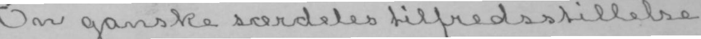En ganske særdeles tilfredsstillelse
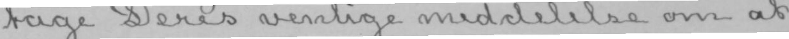tage Deres venlige meddelelse om at
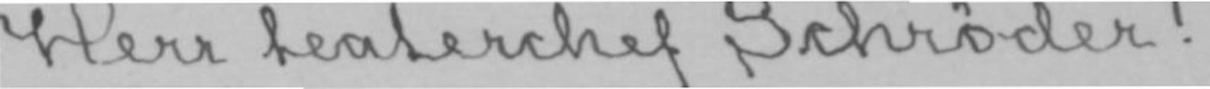Herr teaterchef Schrøder!
